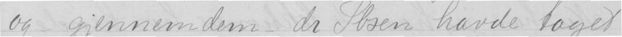og gjennem dem - dr Ibsen havde taget
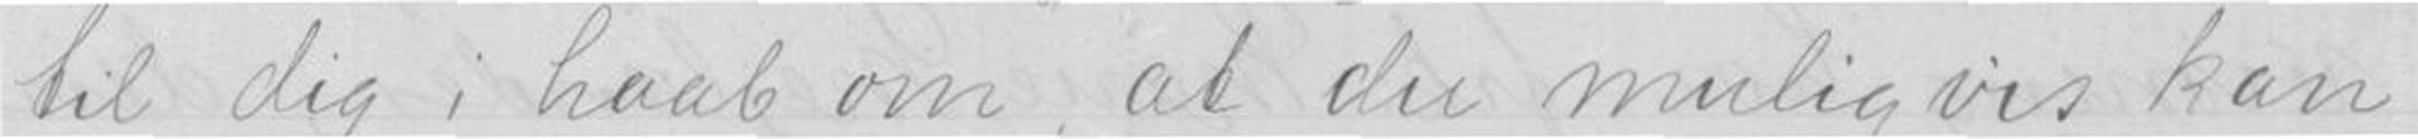til dig i haab om, at du muligvis kan
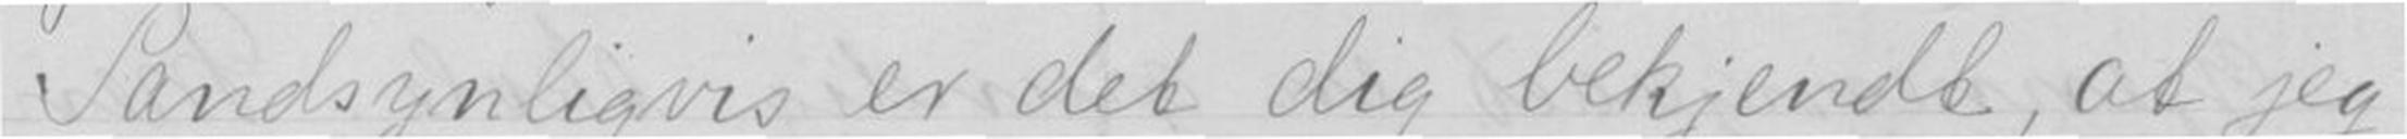Sandsynligvis er det dig bekjendt, at jeg
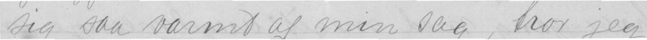sig saa varmt af min sag, tror jeg
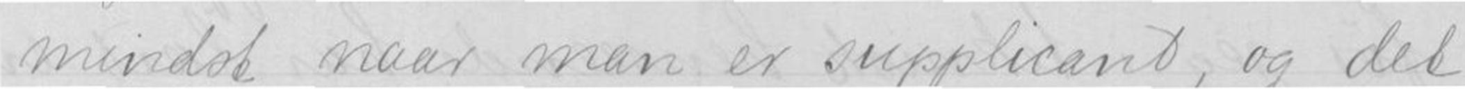mindst naar man er supplicant, og det
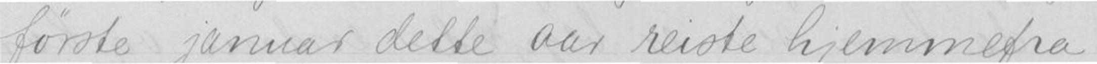første januar dette aar reiste hjemmefra
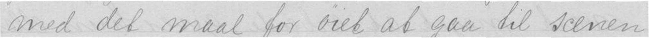med det maal for øiet at gaa til scenen
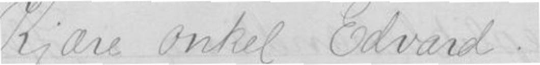Kjære onkel Edvard!
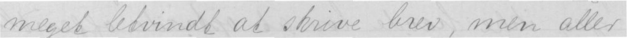meget letvindt at skrive brev, men aller
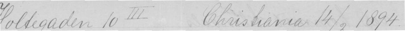Holtegaden 10 III Christiania 14/3 1894.
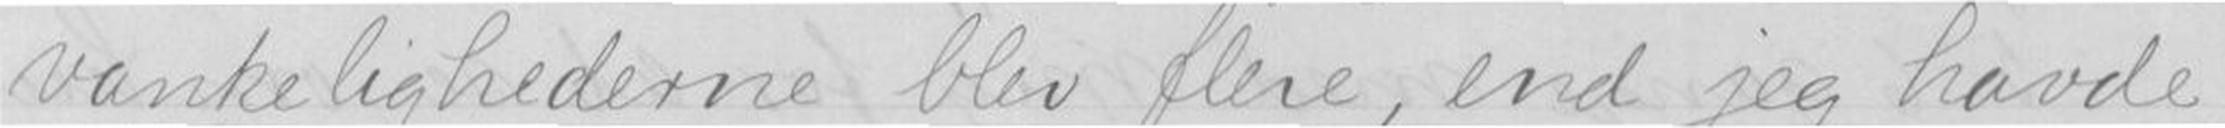vankelighederne blev flere, end jeg havde
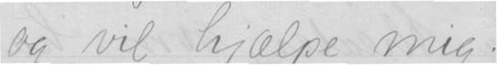og vil hjælpe mig.
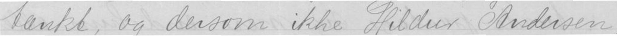tænkt, og dersom ikke Hildur Andersen,
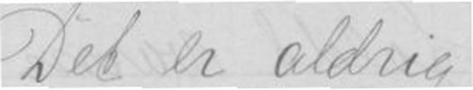Det er aldrig
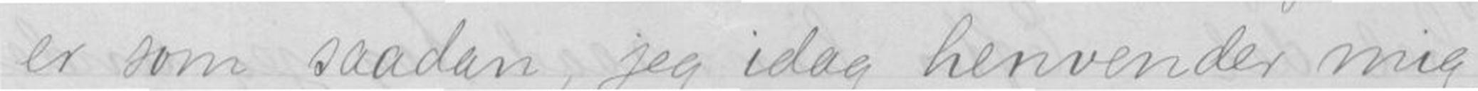er som saadan, jeg idag henvender mig
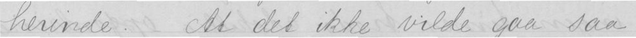herinde. At det ikke vilde gaa saa
